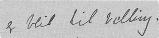er blit til velling.
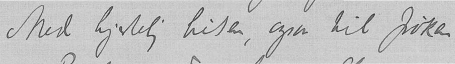Med hjertelig hilsen, ogsaa til frøken
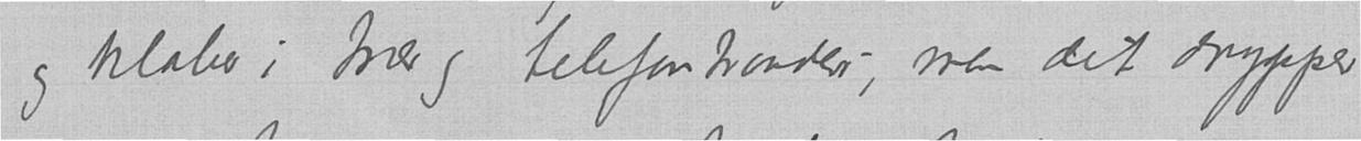og klæber i trær og telefontraader –, men det drypper
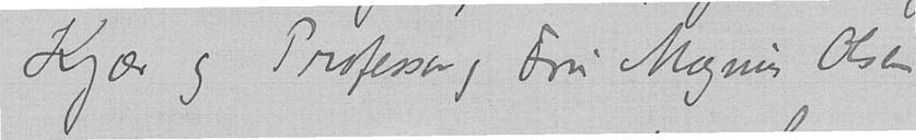Kjær og Professor og Fru Magnus Olsen
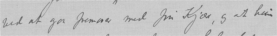ved at gaa fremover med fru Kjær, og at hun
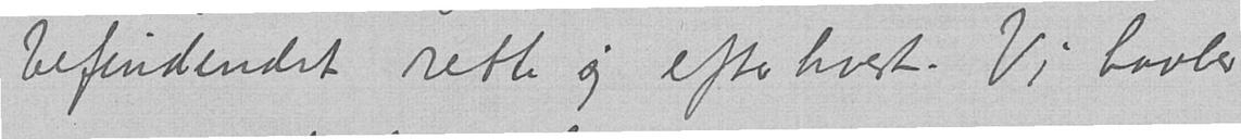befindendet rette sig efterhvert. Vi haaber
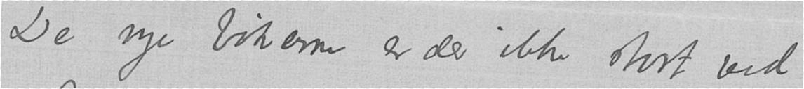De nye bøkerne er der ikke stort ved
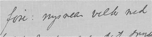føre: nysneen velter ned
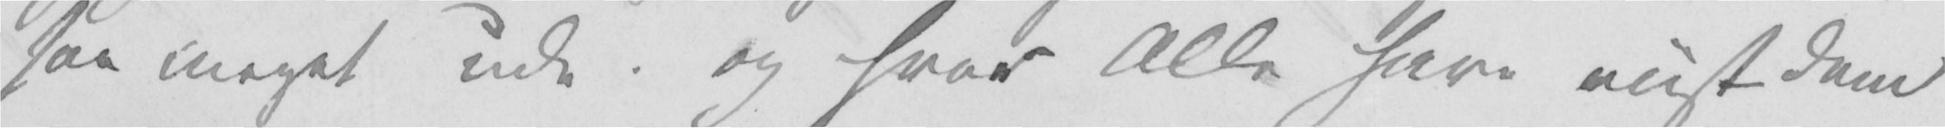saa meget ude. og hvor Alle have vist dem
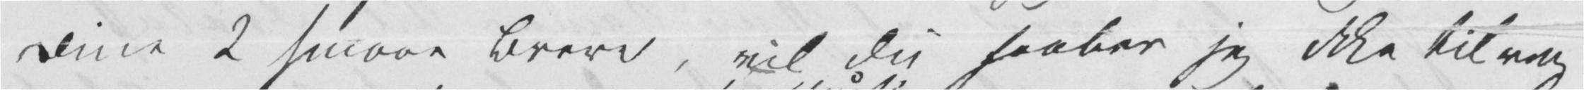Dine 2 smaae Breve, vil Du haaber jeg ikke tilreg
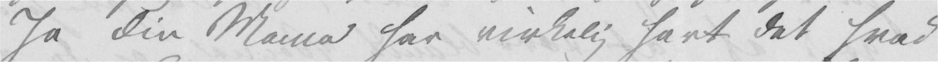Ja din Mama har virkelig havt det hvad
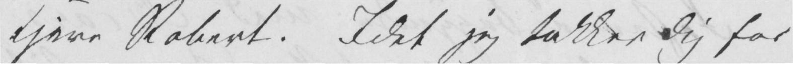Kjere Robert. Idet jeg takker Dig for
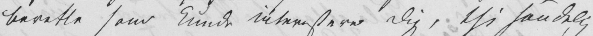berette som kunde interessere Dig, thi sandelig
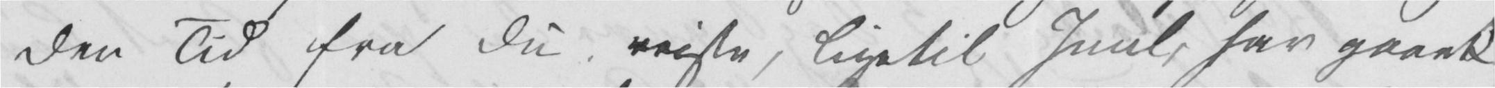den Tid fra Du reiste, ligetil Juul, har gaaet
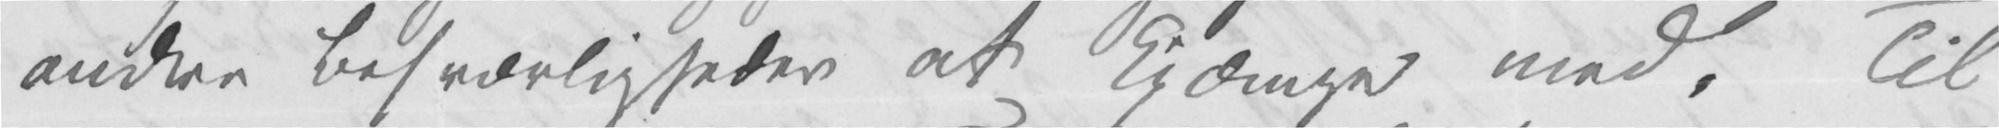andre Besværligheder at kjæmpe med. Til
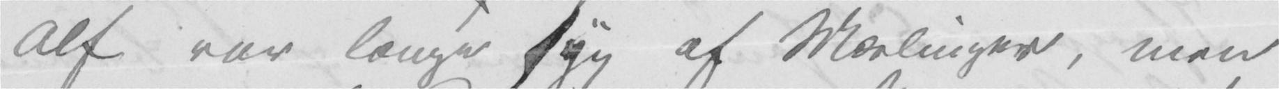Alf var længe syg af Mæslinger, men
